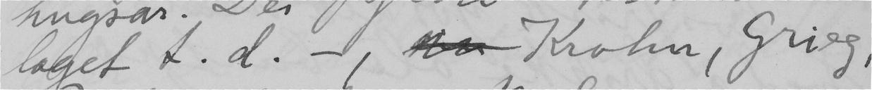laget t.d. –,  Krohn, Grieg,
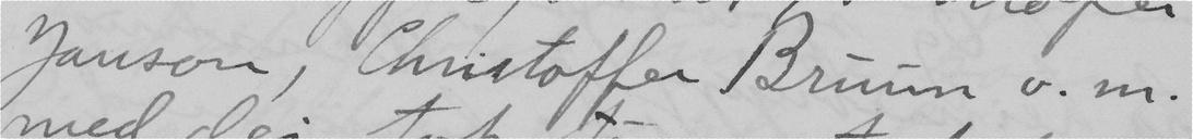Janson, Christoffer Bruun o.m.
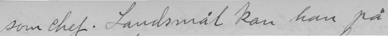som chef. Landsmål kan han på
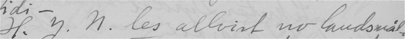H.J.N. les allvist no landsmål-
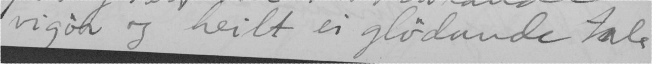vigör og heilt ei glödande Tale
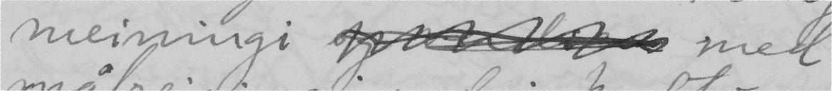meiningi  med
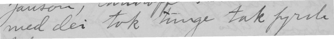med dei tok tunge tak fyrste
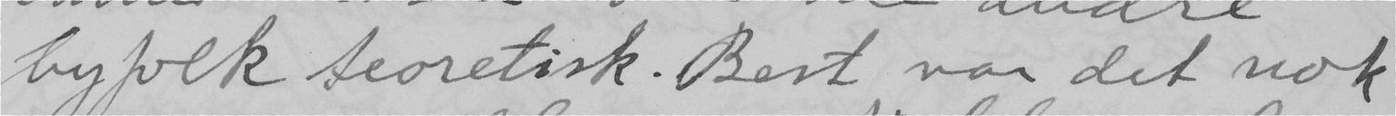byfolk teoretisk. Best var det nok
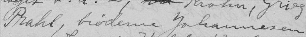Prahl, bröderne Johannesen
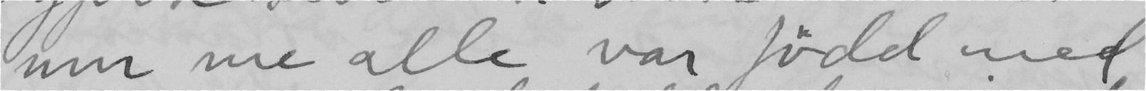um me alle var född med
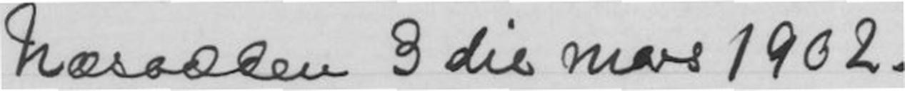Næsodden 3die mars 1902.
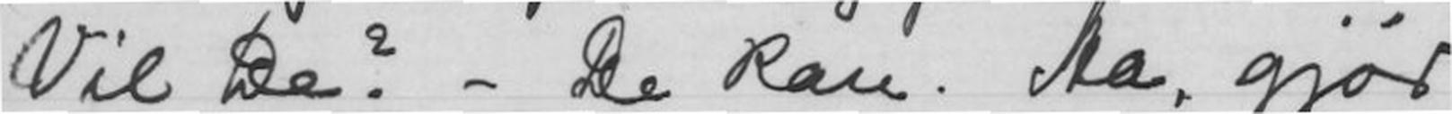Vil De? - De kan. Aa, gjør
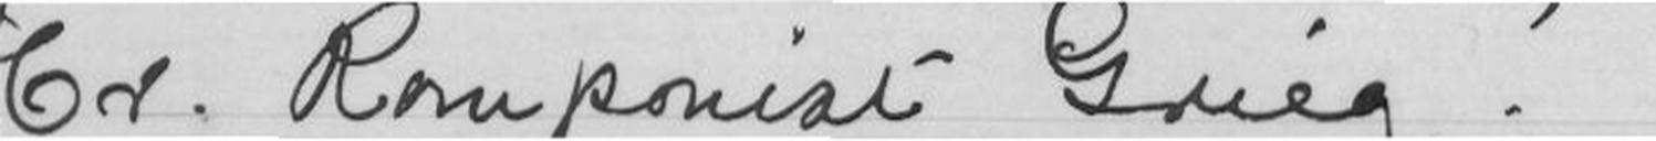Hr. Komponist Grieg!
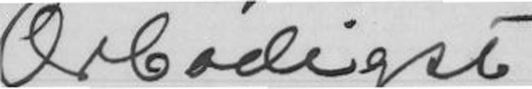Ærbødigst
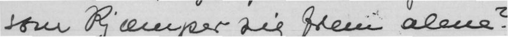som Kjæmper sig frem alene?
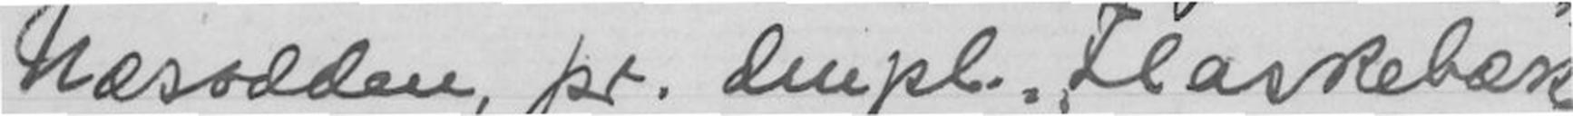Næsodden, pr. denpl. Flaskebæk
